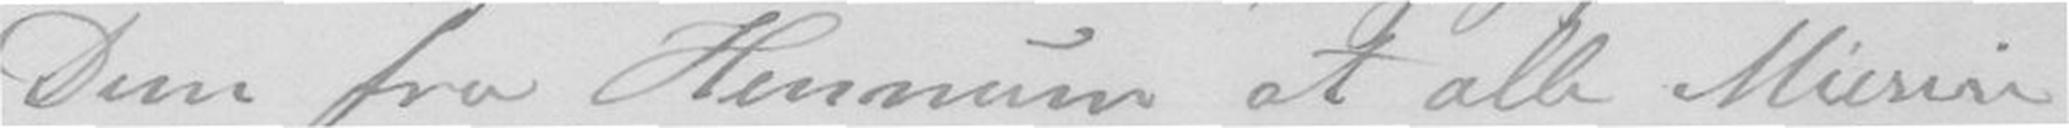Dem fra Hennum at alle Museu
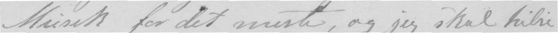Musik for det meste, og jeg skal hilse
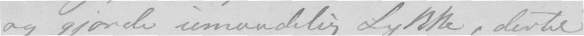og gjorde umaadelig Lykke, dertil
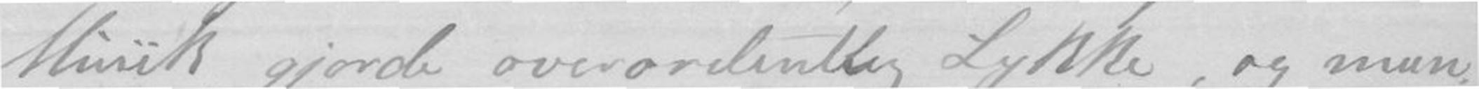Musik gjorde overordentlig Lykke, og man-
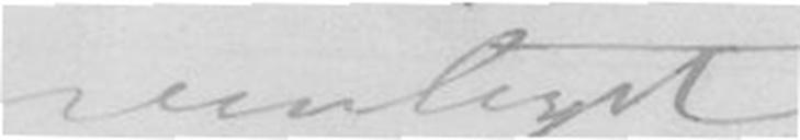venligst
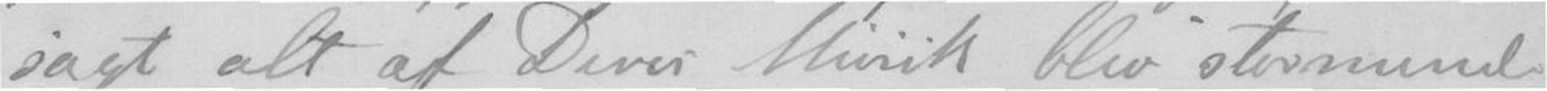sagt alt af Deres Musik blev stormende
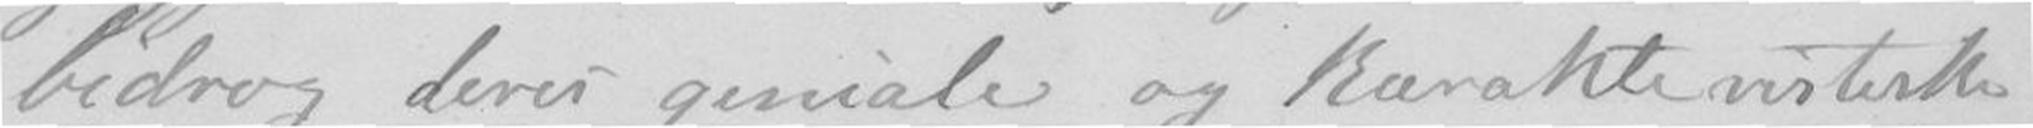bidrog deres geniale og karakteristiske
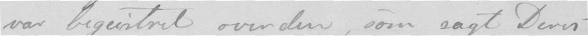var begeistret over den, som sagt Deres
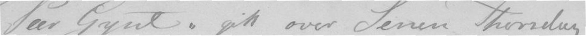Peer Gyndt" gik over Senen Thorsdag
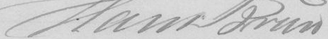Hans Brun
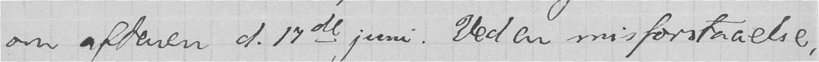om aftenen d. 17de juni. Ved en misforstaaelse,
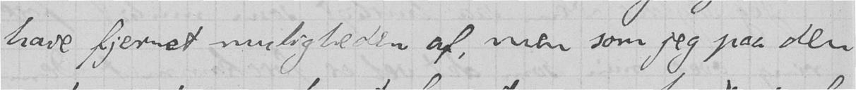have fjernet muligheden af, men som jeg paa den
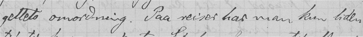gettets omordning. Paa reiser har man kun liden
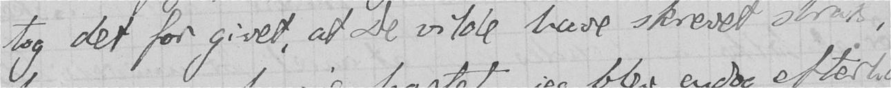tog det for givet, at De vilde have skrevet strax,
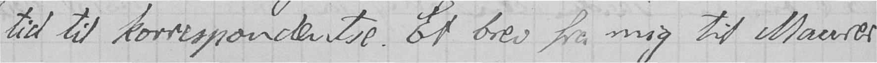tid til korrespondentse. Et brev fra mig til Maurer
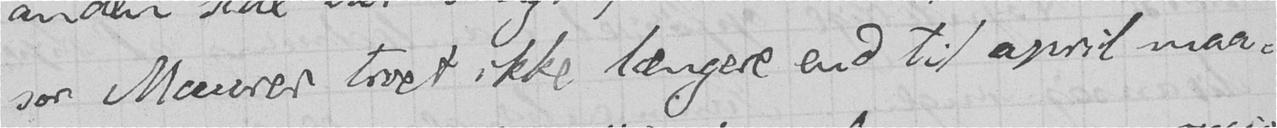sor Maurer troet ikke længere end til april maa-
